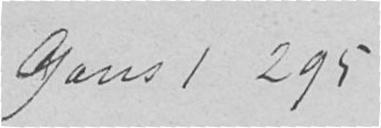Gans I 295
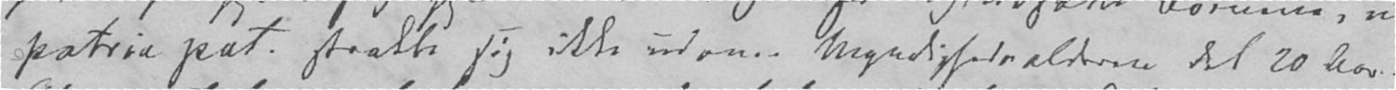Patria Pot. strakte sig ikke udover Myndighedsalderen det 20 Aar.
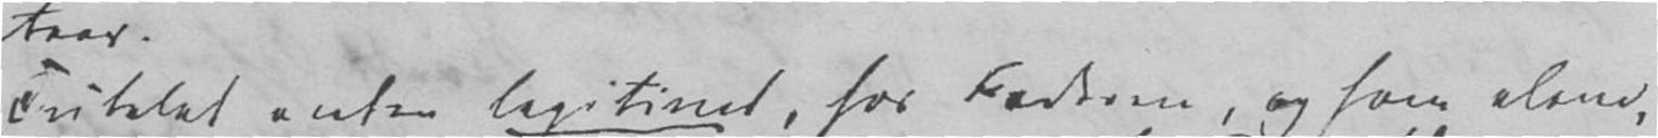Tutelat enten legitimt, hos Faderen, og ham alene,
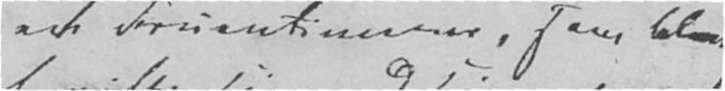et Fruentimener, som blev
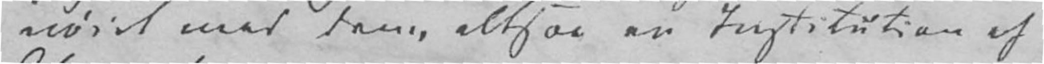nøiet med dem, altsaa en Institution af
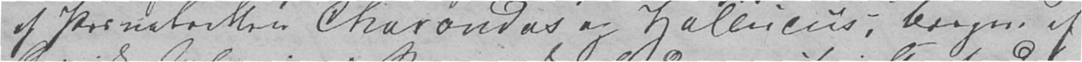af Privatretten Charondas og Hallicus, Borgere af
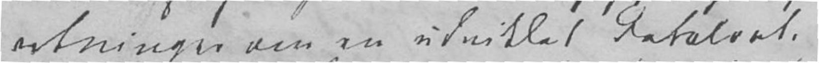retninger om en udviklet Dotalret.
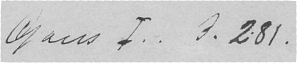Gans I. 3. 281.
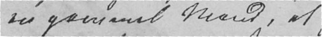en gammel Mand, at
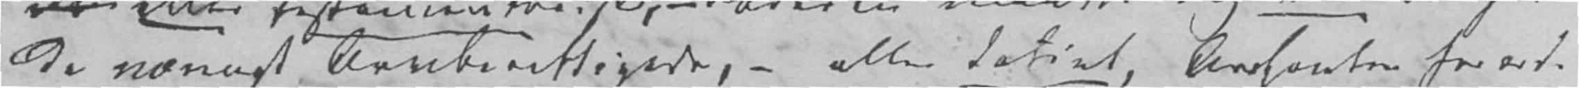de nærmest Arveberettigede, - eller dotiet, Archonten forord-
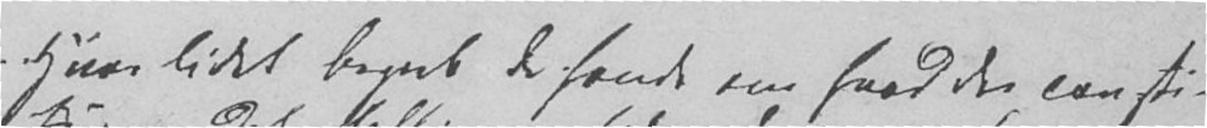Hvor lidet Begreb de havde om hvad der consti-
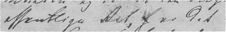offentlige Ret, h er det
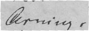Arving,
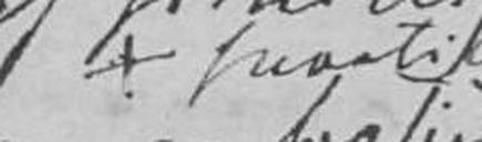hvortil
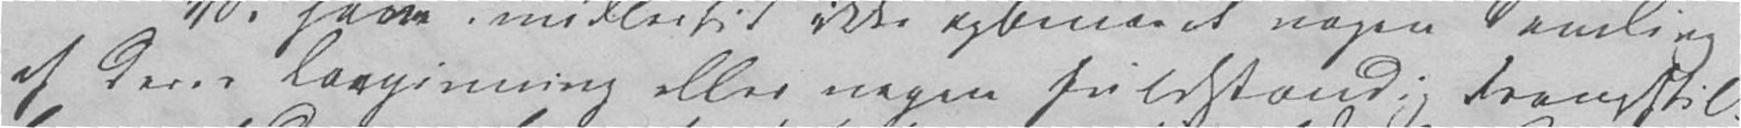af deres Lovgivning eller nogen fuldstændig Framstil-
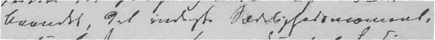baandet, det inderste Sædelighedsmoment,
